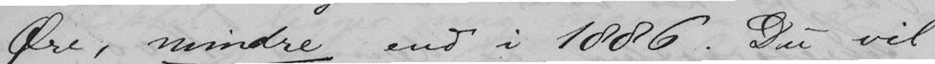Øre, mindre end i 1886. Du vil
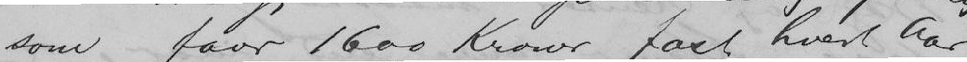som faar 1600 Kroner fast hvert Aar
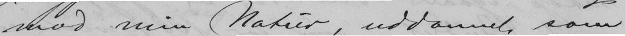mod min Natur, uddannet som
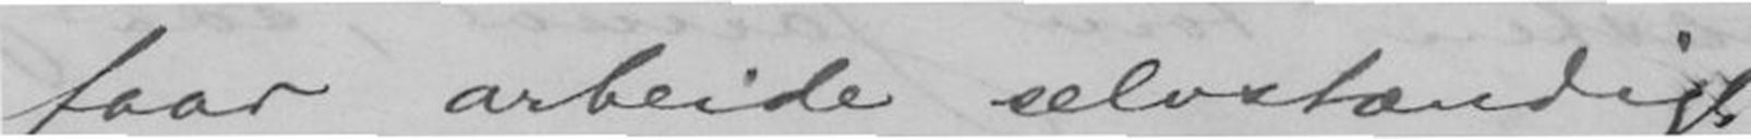faar arbeide selvstendigt
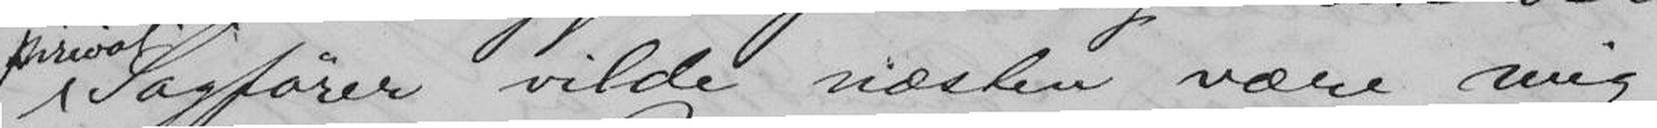Sagfører vilde næsten være mig
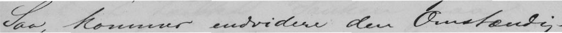Saa kommer endvidere den Omstændig-
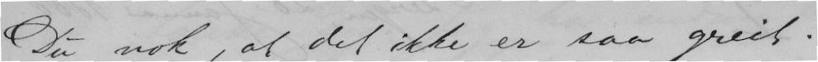Du nok, at det ikke er saa greit.
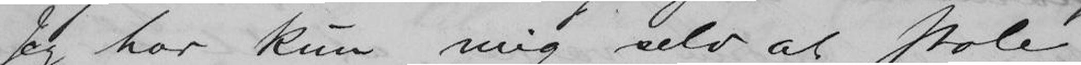Jeg har kun mig selv at stole
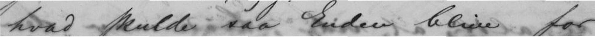hvad skulde saa Enden blive for
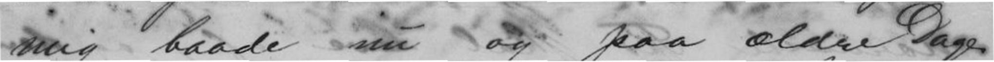mig baade nu og paa ældre Dage.
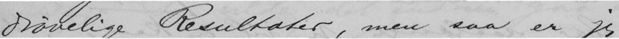drøvelige Resultater, men saa er jeg
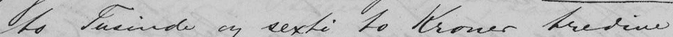to Tusinde og sexti to Kroner tredive
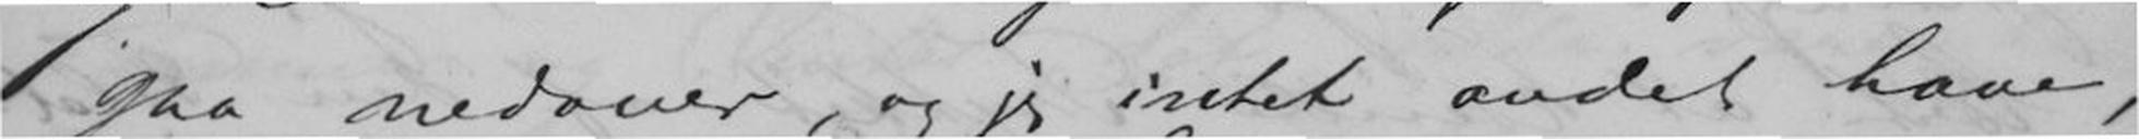gaa nedover, og jeg intet andet have,
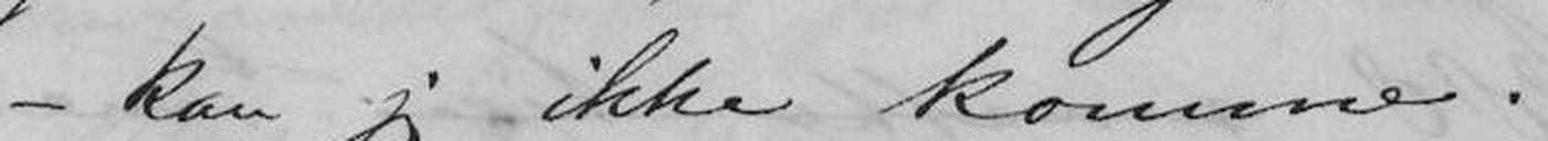-Noter: g! - kan jeg ikke komme.
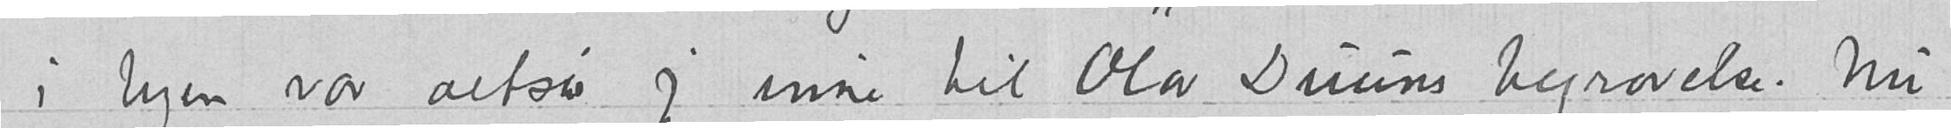i byen var altså jeg inne til Olav Duuns begravelse. Nu
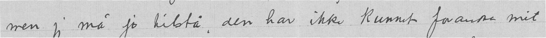men jeg ma jo tilstå, den har ikke kunnet forandre mit
